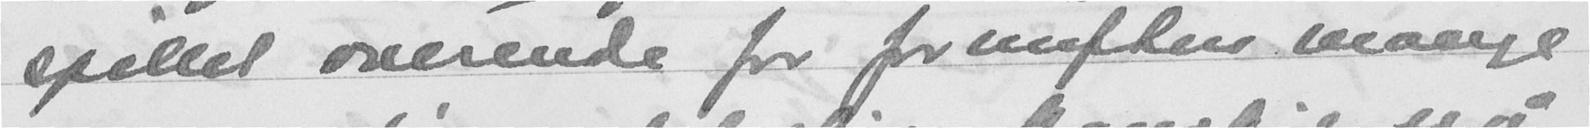spillet overende for fornuften mange
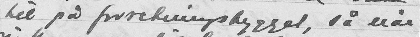til på forretningsbygget, så når
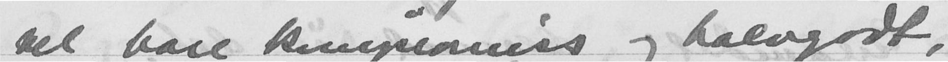vel bare kompromis og halvgodt,
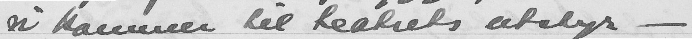vi kommer til teatrets utstyr -
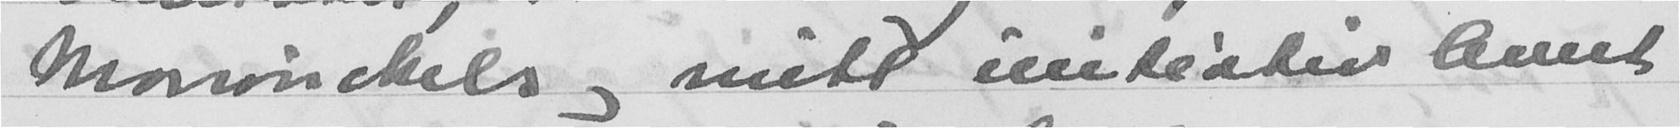Mowinckels og mitt initiativ lavet
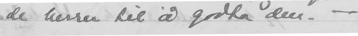de herrer til å godta den. -
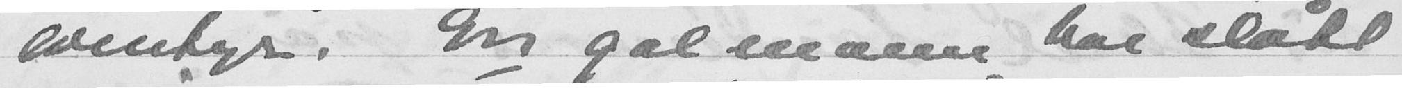eventyr. En gal mann har slått
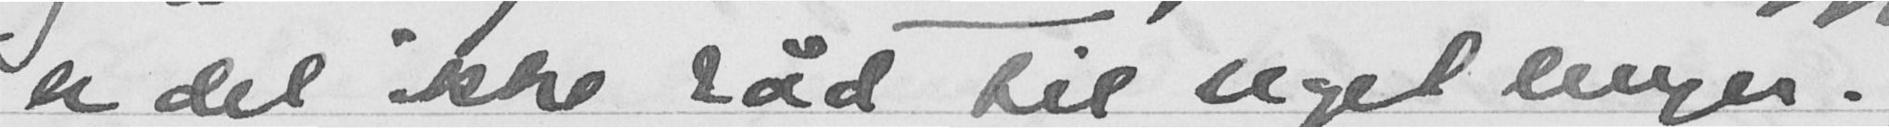er det ikke råd til meget lenger.
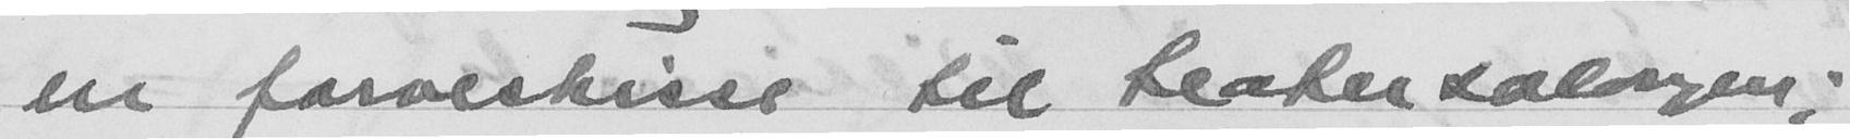en farveskisse til teatersalongen,
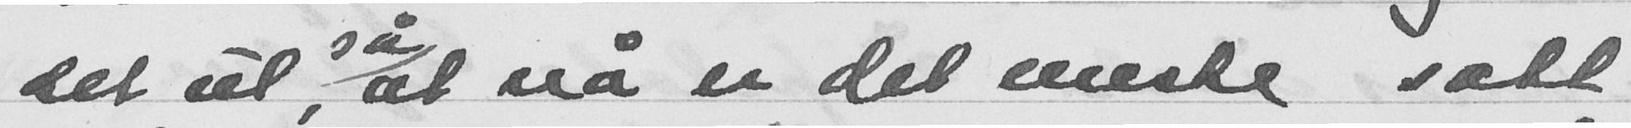det ut, så/at nå er det meste satt
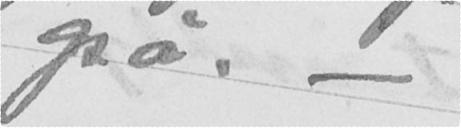også. -
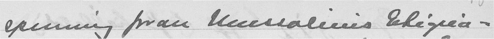spenning foran Mussolinis Etiopia-
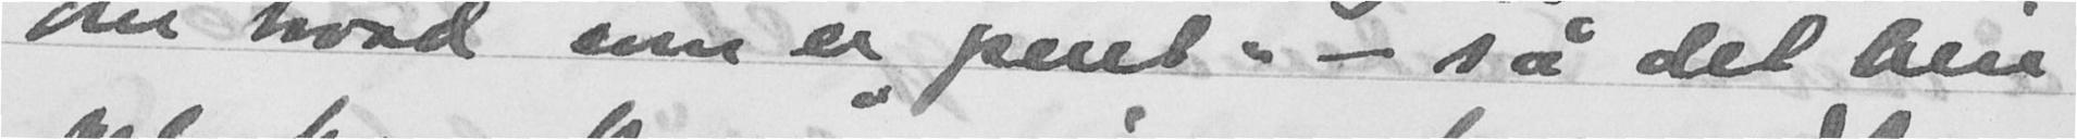om hvad som er "pent" - så det blir
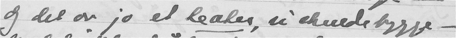og det er jo et teater, vi skulde bygge -
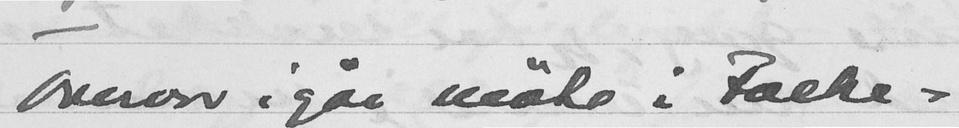Oveverv igår møte i Folke-
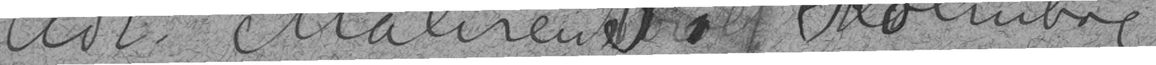Adr: Maleren TorolfT. Holmboe
